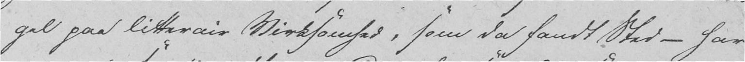gel paa litterair Virksomhed, som da fandt Sted - har
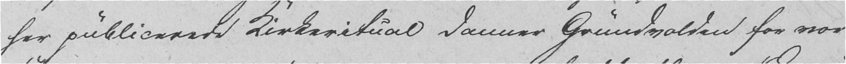her publicerede Kirkeritual danner Grundvolden for vor
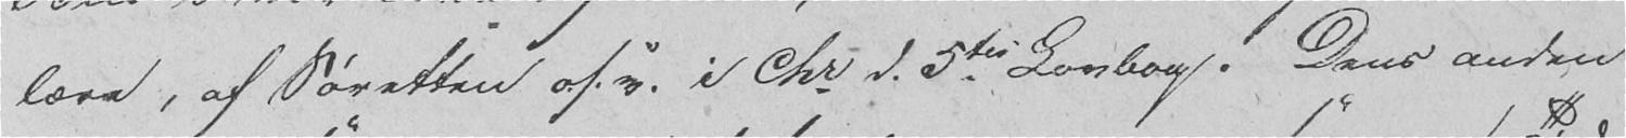lære, af Søretten o. s. v. i Chr d. 5tes Lovbog. Dens anden
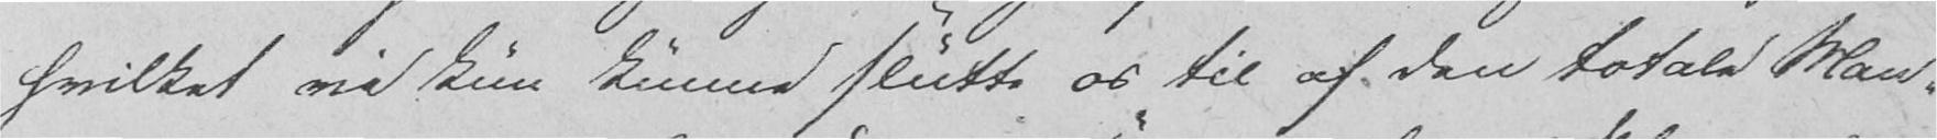hvilket vi kun kunne slutte os til af den totale Man-
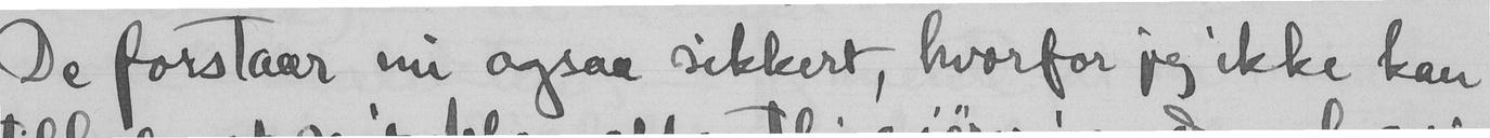De forstaar nu ogsaa sikkert, hvorfor jeg ikke kan
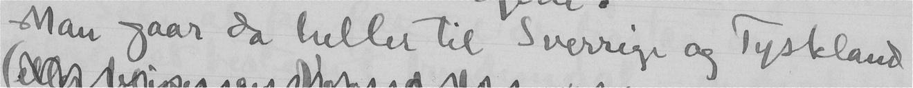Man gaar da heller til Sverrige og Tyskland
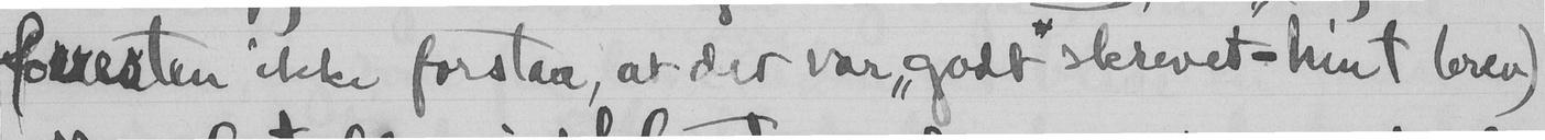forresten ikke forstaa, at det var "godt skrevet" - hint brev)
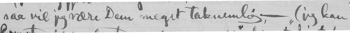saa vil jeg være Dem meget taknemlig - (jeg kan
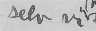selv vi
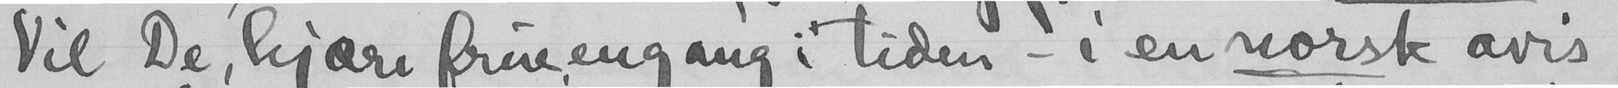Vil De, kjære frue engang i tiden - i en norsk avis
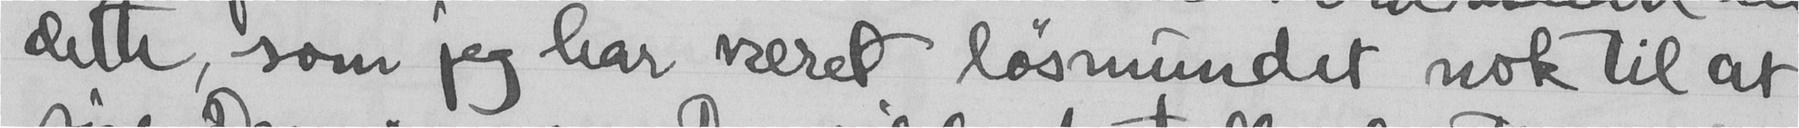dette som jeg har været løsmundet nok til at
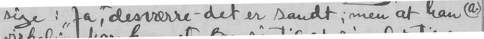sige: "Ja", desværre - det er sandt,; men at han (a)
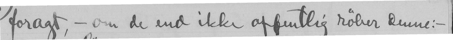foragt, - om de end ikke offentlig røber denne; -
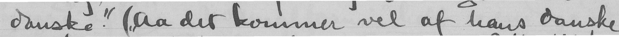danske". ("Aa det kommer vel af hans danske
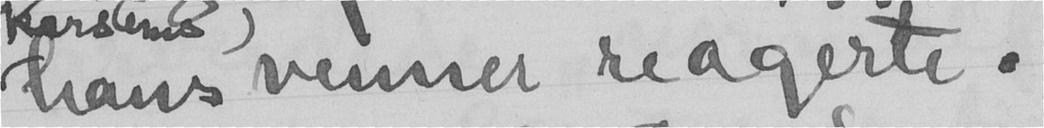hans venner reagerte.
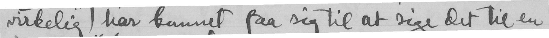virkelig, har kunnet faa sig til at sige det til en
